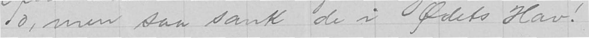o, men saa sank de i Ødets Hav!
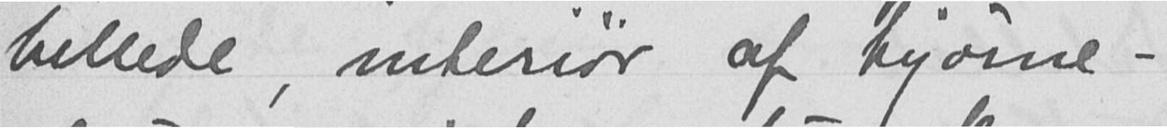billede, interiør af hjørne-
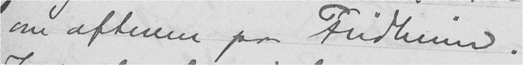om aftenen paa Fridheim.
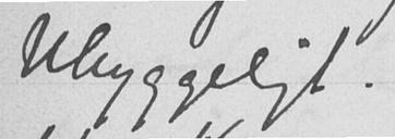Uhyggeligt.
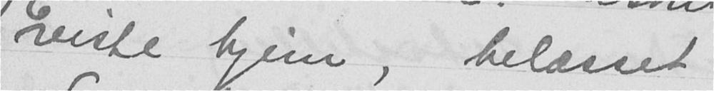reiste hjem, belæsset
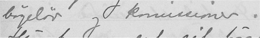bøgeløv og komissioner.
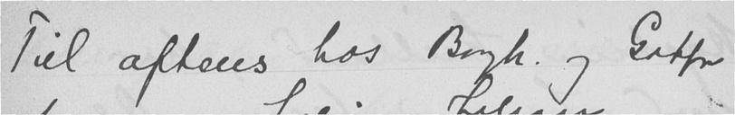Til aftens hos Borgh. og Gotfr
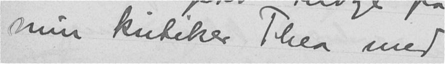min kritiker Thea med
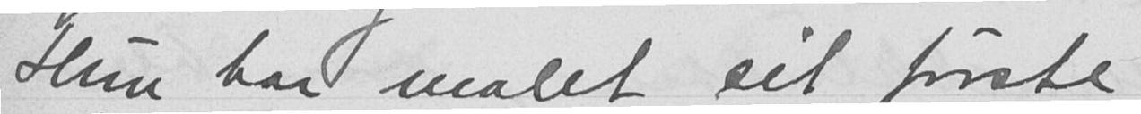Hun har malet sit første
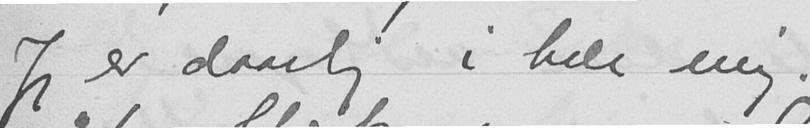Jeg er daarlig i hele mig.
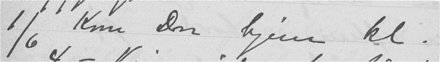1/6 Kom Don hjem kl.
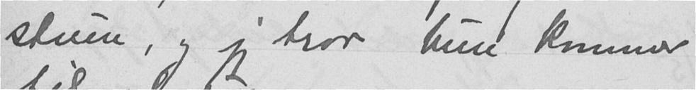stuen, og jeg tror hun kommer
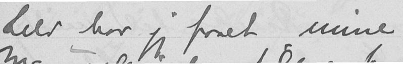held har jeg faaet mine
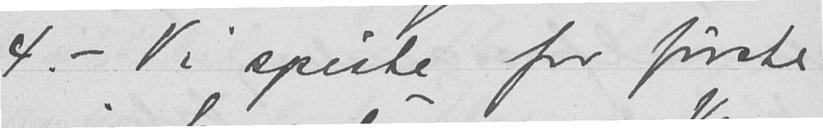4. - Vi spiste for første
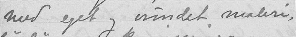med eget og vundet maleri,
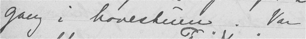gang i hovestuen. Var
